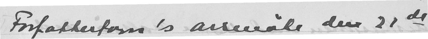Forfattesforn's årsmøte den 21de
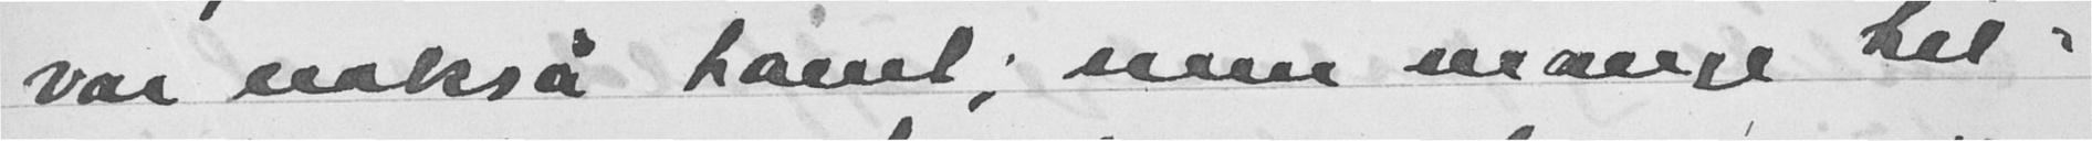var nokså tamt; men mange til-
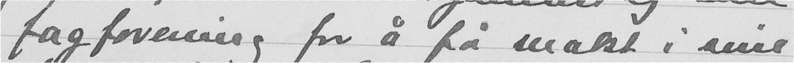fagforening for i få makt i sine
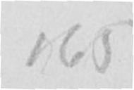165
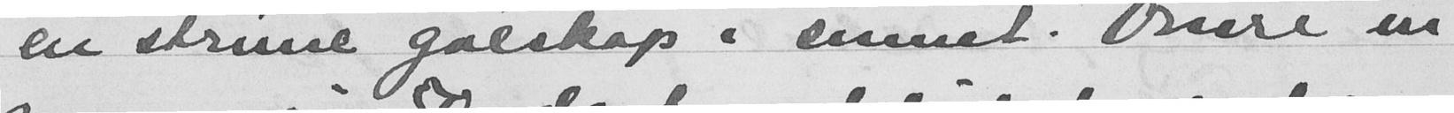en strime galskap i sinnet. Omre en
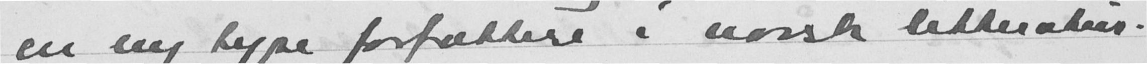en ny type forfattere i norsk litteratur.
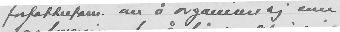forfatterforn. om å organisere sig som
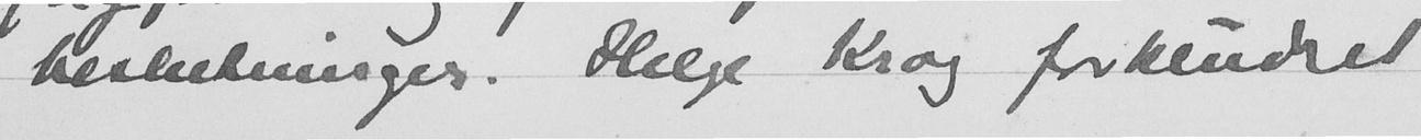beslutninger. Helge Krog forkludret
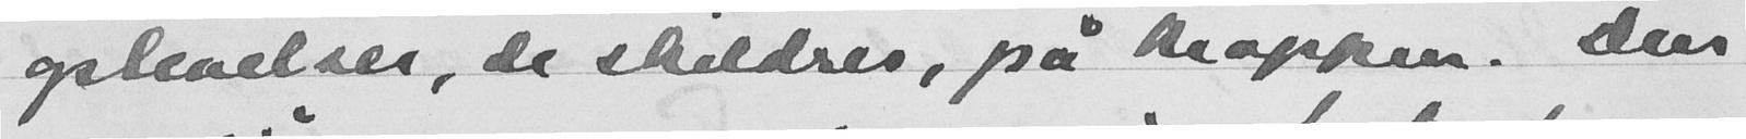oplevelser, de skildrer, på kroppen. Den
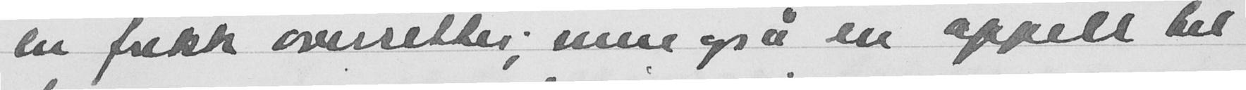en frekk oversetter; men også en appell til
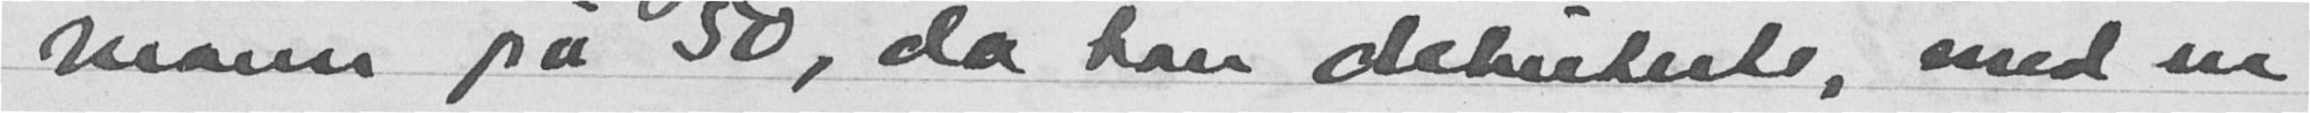mann på 50, da han debuterte, med en
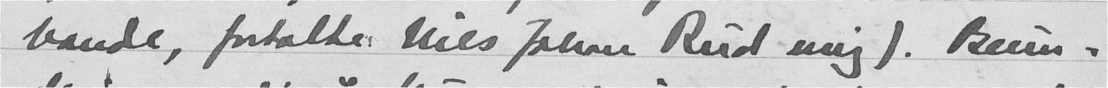bande, fortalte Nils Johan Rud mig). Beun-
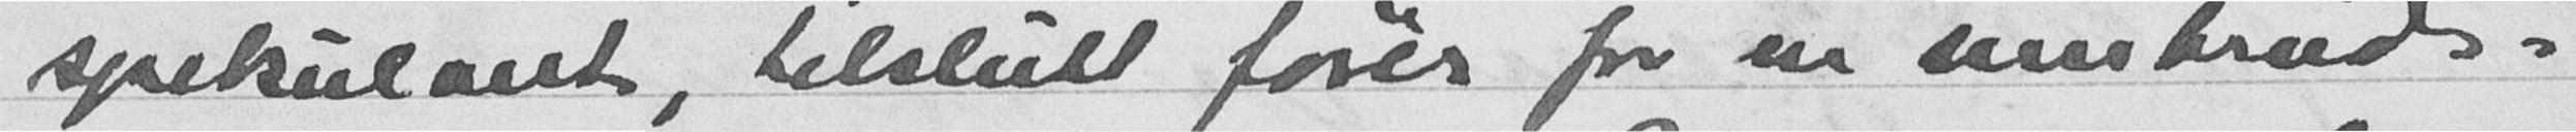spekulant, tilslutt fører for en innsbruds-
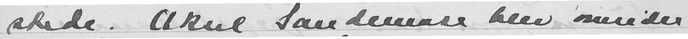stede. Aksel Sandemose blir omsider
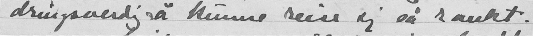dringsverdig å kunne reise sig så rankt.
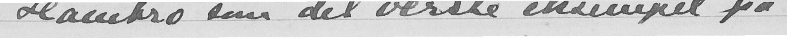Hambro som det verste eksempel på
